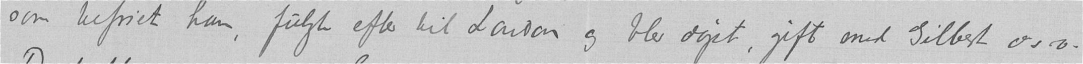som befriet ham, fulgte efter til London og blev døpet, gift med Gilbert osv.
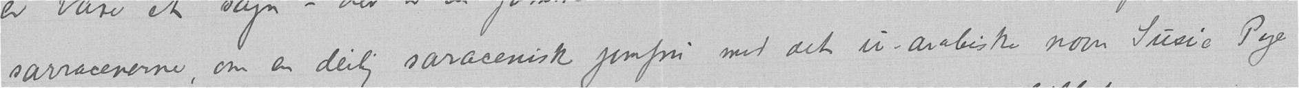sarracenerne, om en deilig saracenisk jomfru med det u-arabiske navn Susie Page
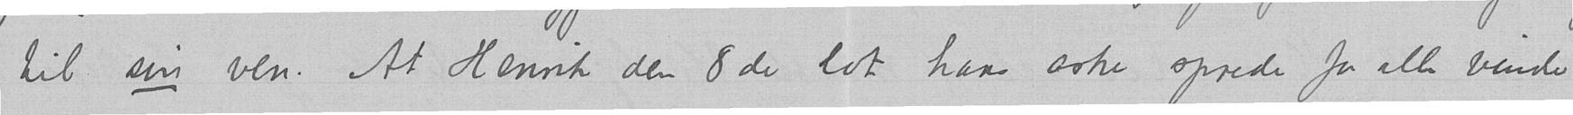til sin ven. At Henrik den 8de lot hans aske sprede for alle vinde
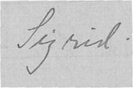Sigrid.
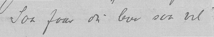Saa faar du leve saa vel!
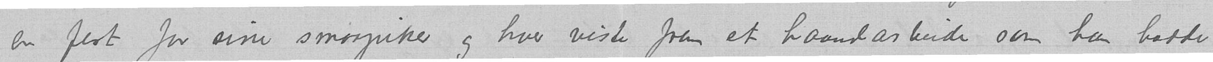en fest for sine smaapiker og hver viste frem et haandarbeide som han hadde
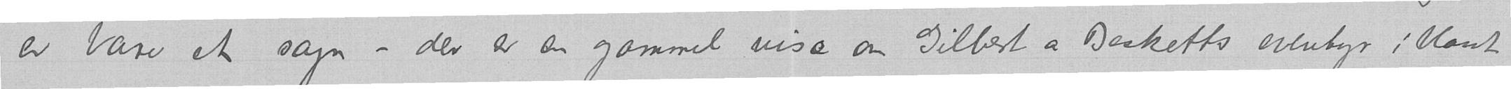er bare et sagn – der er en gammel vise om Gilbert a Becketts eventyr i blant
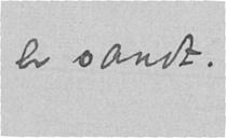er sandt.
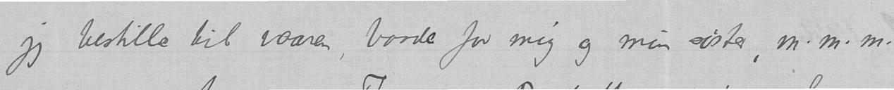jeg bestille til vaaren, baade for mig og min søster, m.m.m.
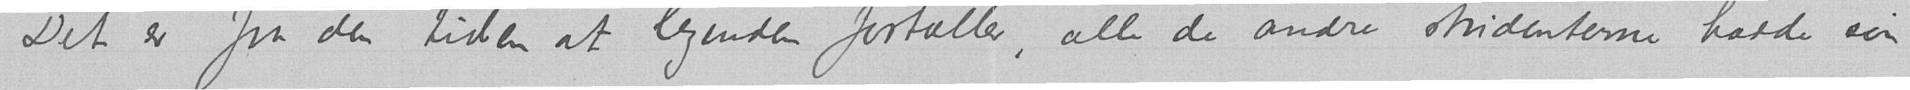Det er fra den tiden at legenden fortæller, alle de andre studenterne hadde sin
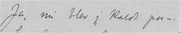Ja, nu blev jeg kaldt paa –.
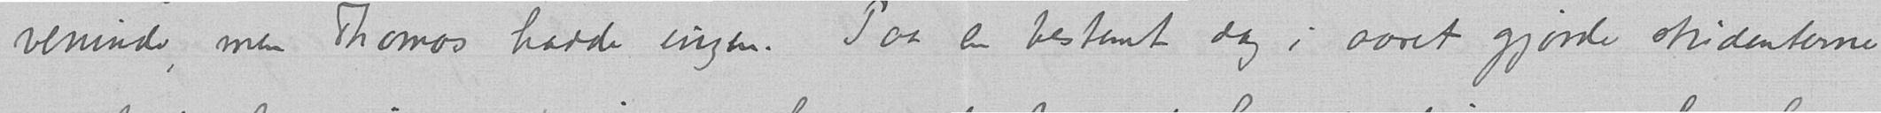veninde, men Thomas hadde ingen. Paa en bestemt dag i aaret gjorde studenterne
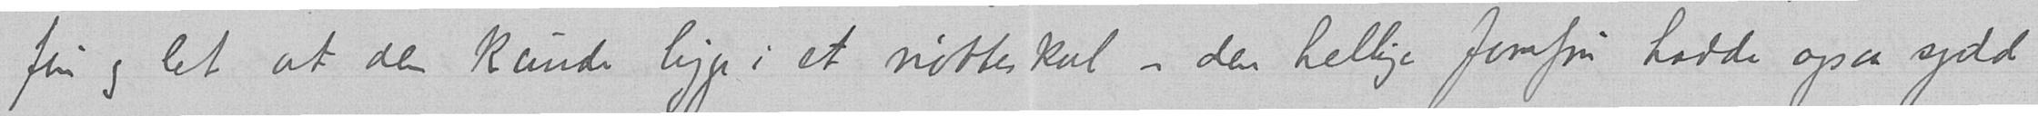fin og let at den kunde ligge i et nøtteskal – den hellige jomfru hadde ogsaa sydd
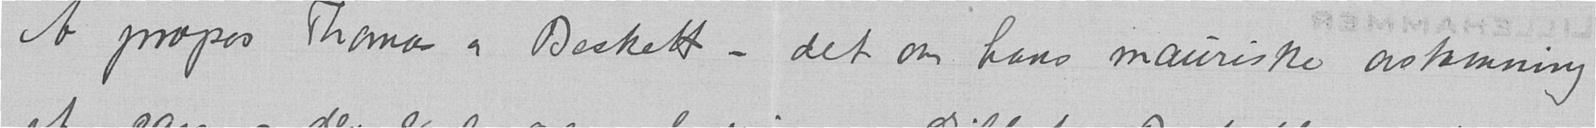A propos Thomas a Beckett – det om hans mauriske avstamning
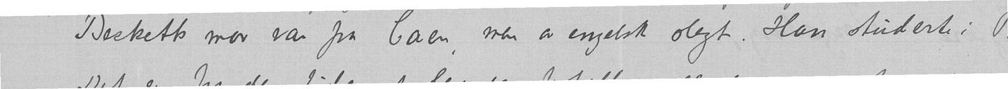Becketts mor var fra Caen, men av engelsk slegt. Han studerte i Paris.
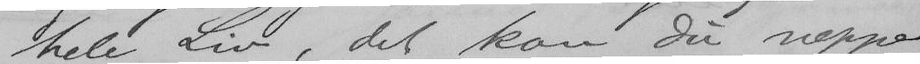hele Liv, det kan Du neppe
